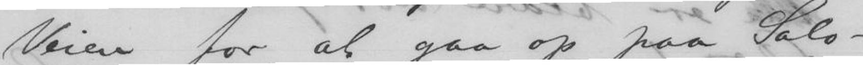Veien for at gaa op paa Salo-
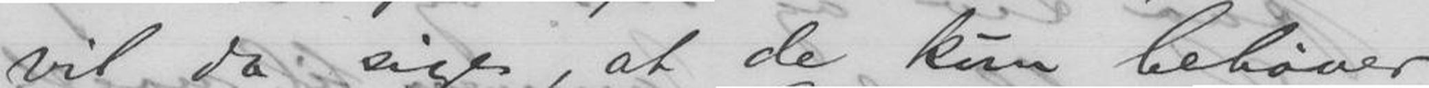vil da sige, at de kun behøver
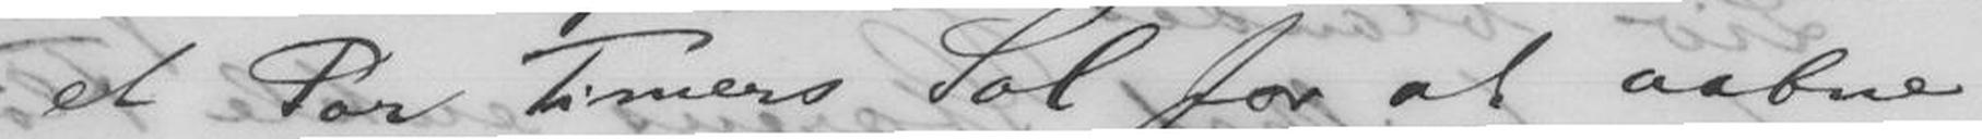et Par Timers Sol for at aabne
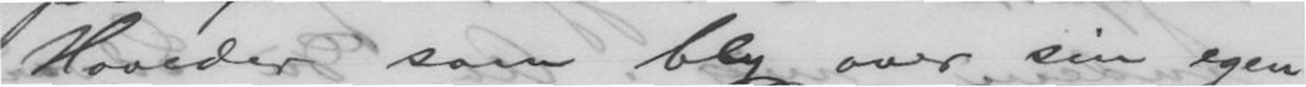Hoveder som bly over sin egen
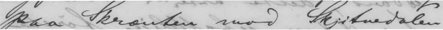paa Skrænten mod Skjitnedalen
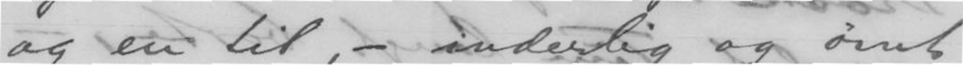og en til, - inderlig og ømt
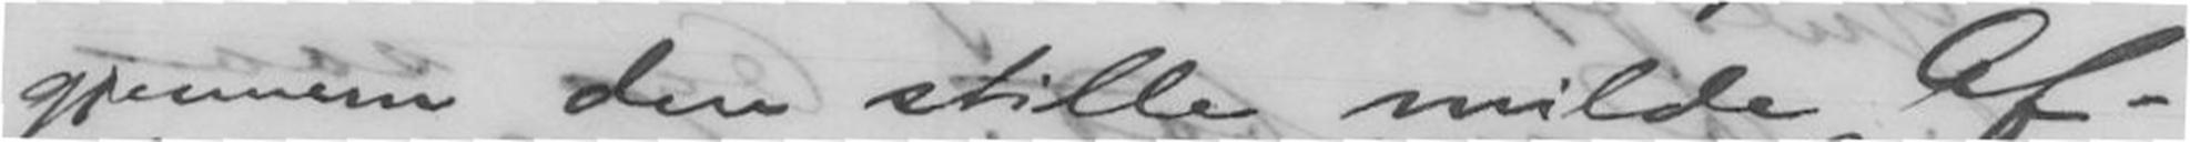gjennem den stille milde Af-
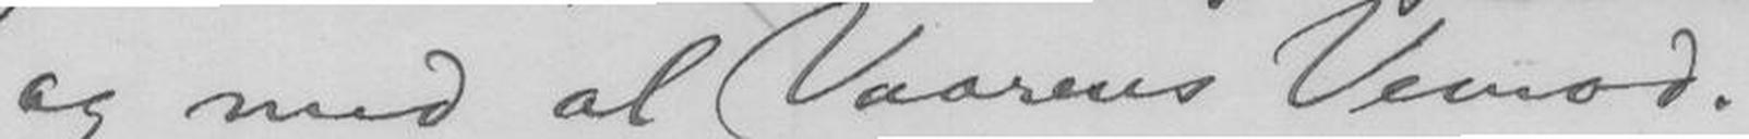og med al Vaarens Vemod.
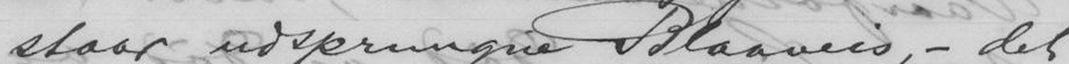staar udsprungne Blaaveis, - det
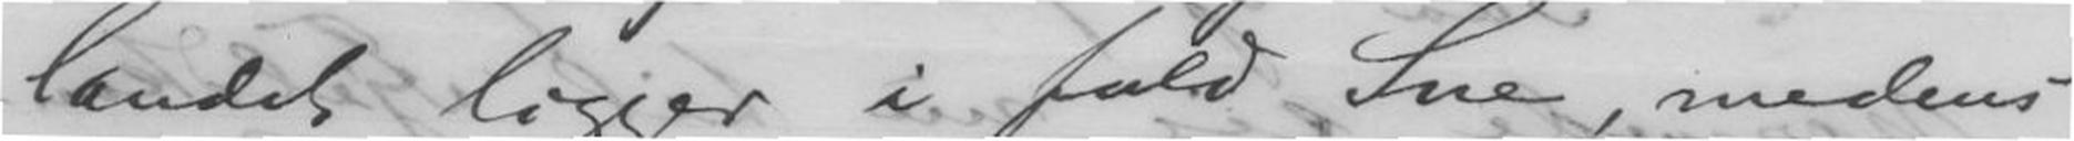landet ligger i fuld Sne, medens
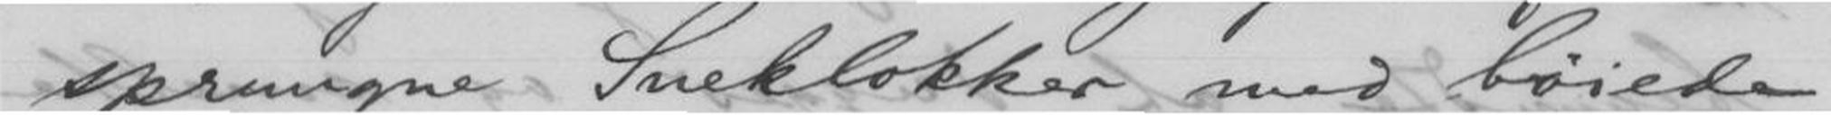sprungne Sneklokker med bøiede
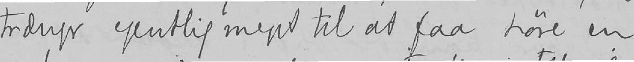trænger egentlig meget til at faa høre en
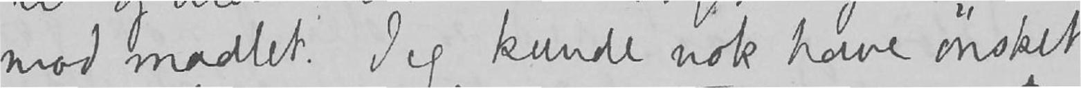mod maalet. Jeg kunde nok have ønsket
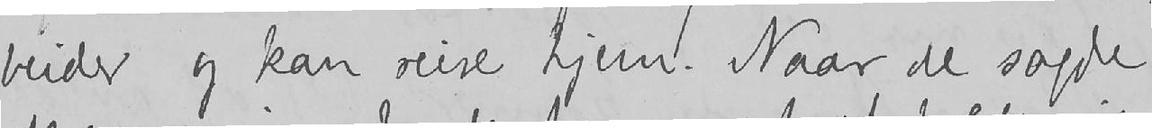beider og kan reise hjem. Naar de sagde
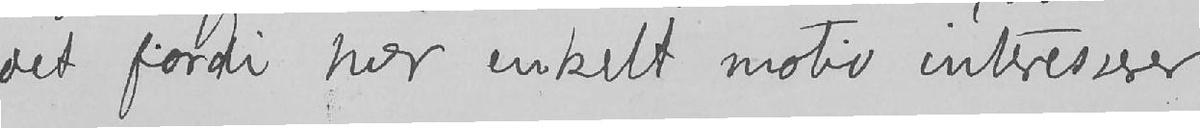det fordi hver enkelt motiv interesserer
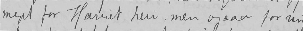meget for Harriet heri, men ogsaa for mig
